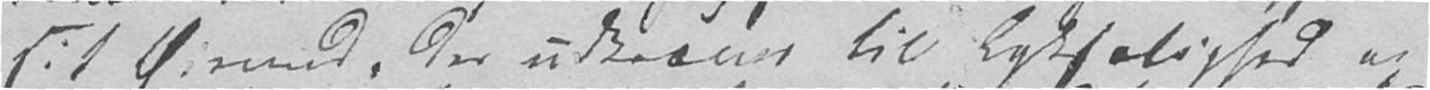sit Øiemed, der udkræves til Lyksalighed og
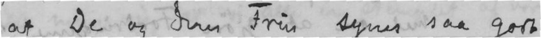at De og Deres Frue synes saa godt
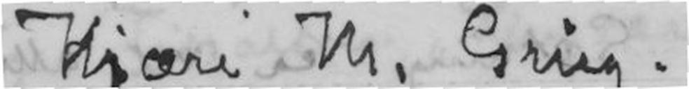Kjære Hr. Grieg.
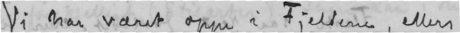Vi har været oppe i Fjeldene, ellers
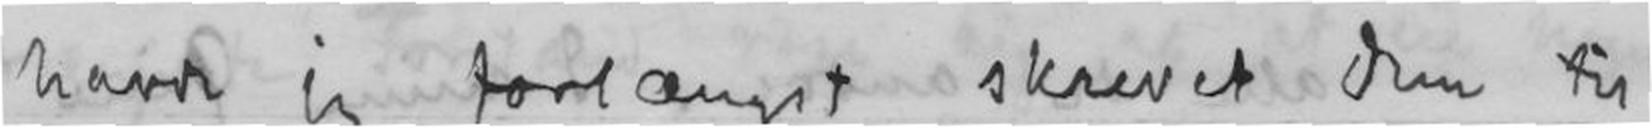havde jeg forlængst skrevet Dem til
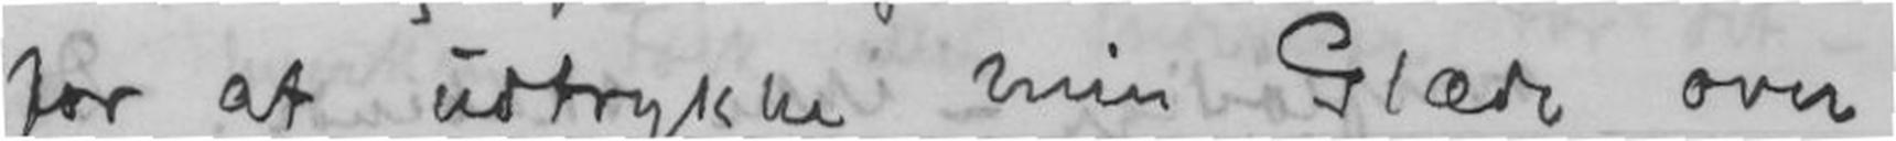for at udtrykke min Glæde over
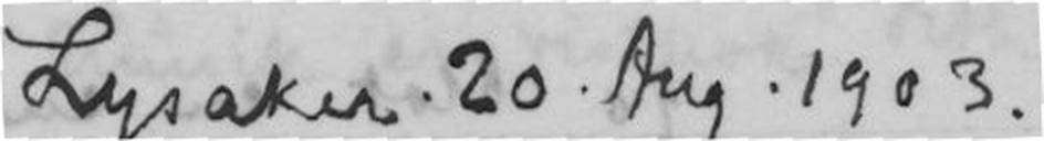Lysaker. 20. Aug. 1903.
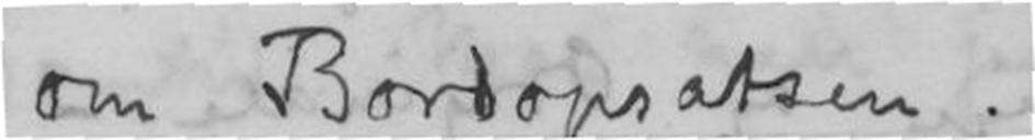om Bordopsatsen.
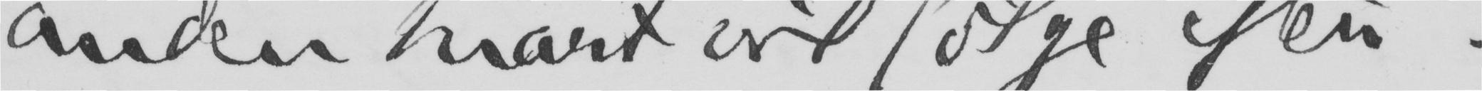anden snart vil følge efter.
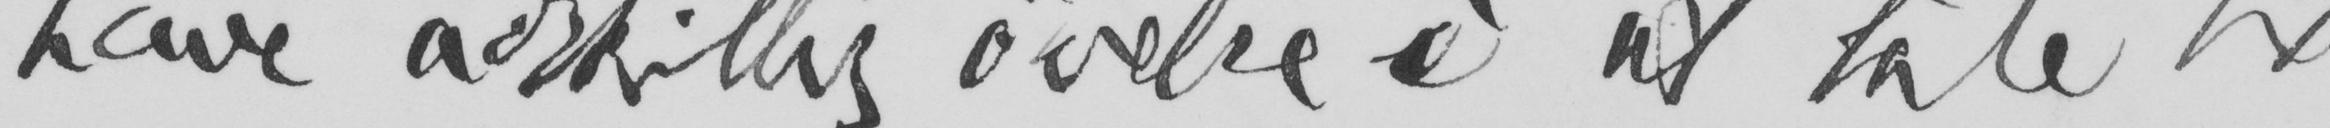have adskillig øvelse i at tale til.
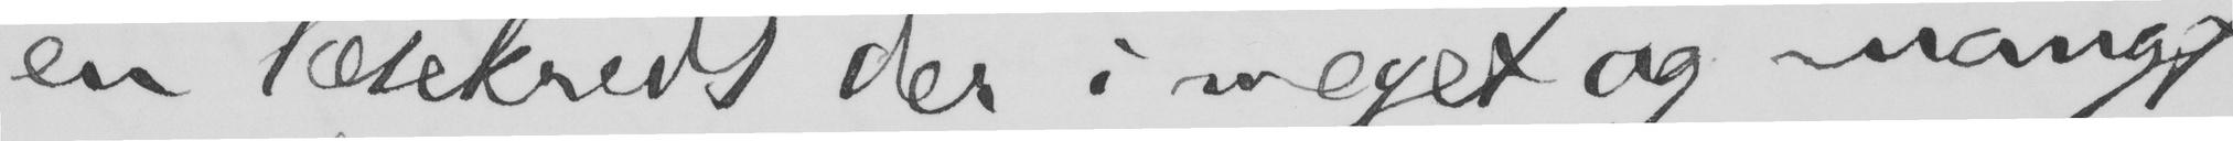en læsekreds der i meget og mangt
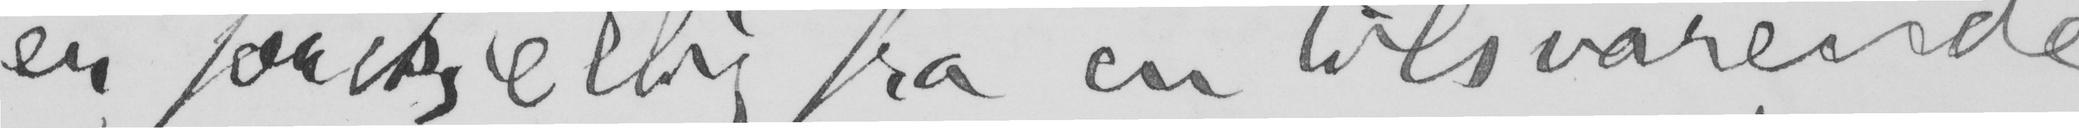er forskjellig fra en tilsvarende
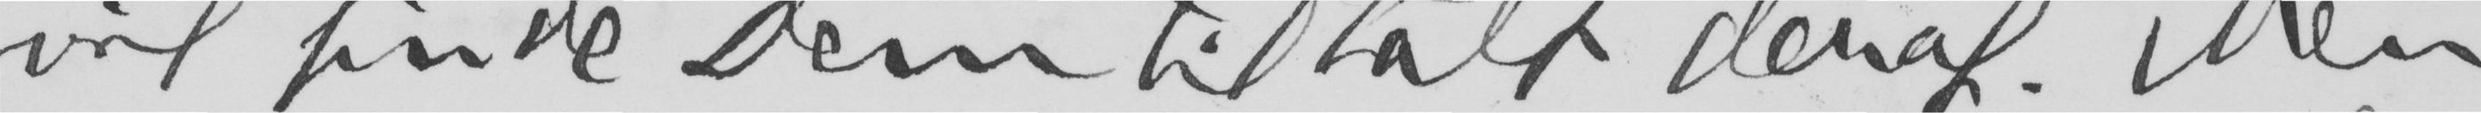vil finde Dem tiltalt deraf. Men
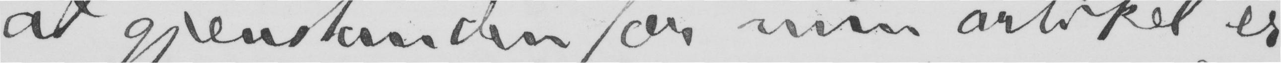at gjenstanden for min artiklel er
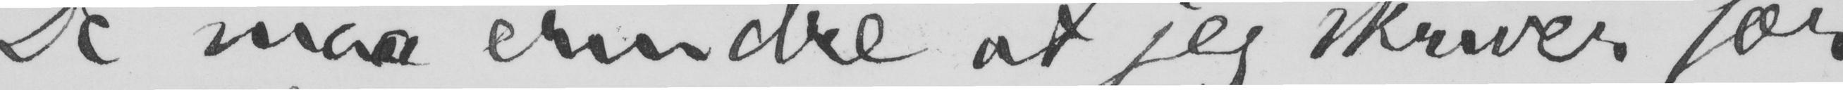De maa erindre, at jeg skriver for
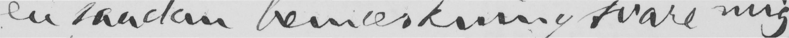en saadan bemærkning svare mig,
